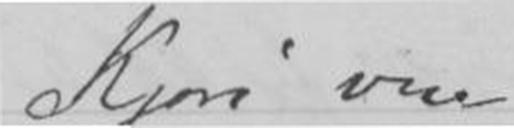Kjære ven
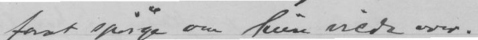forat spørge øm hun vilde over-
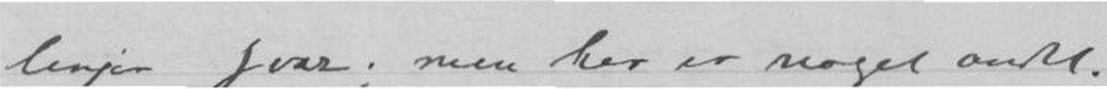linjer svar; men her er noget andet.
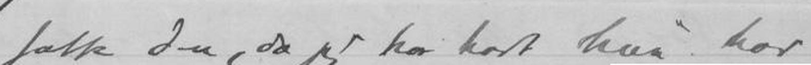sætte den, da jeg har hørt hun har
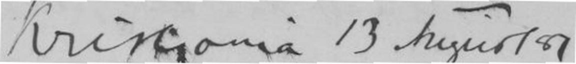Kristiania 13 August 81
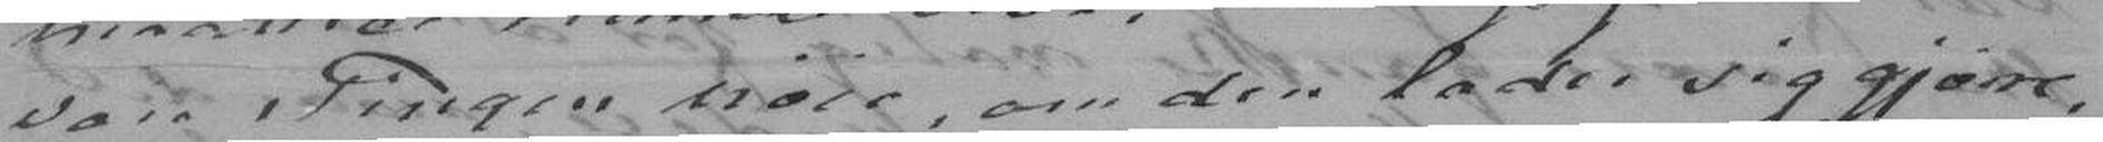veie Tingen nøie, om den lader sig gjøre,
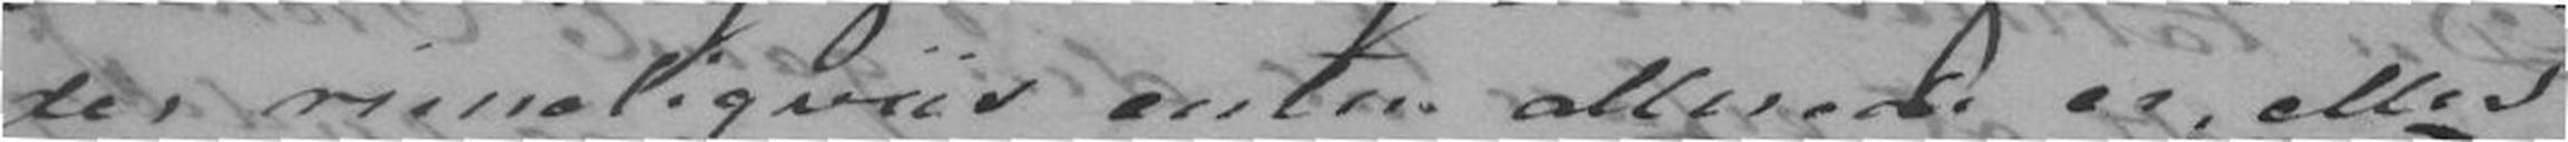der rimeligviis enten allerede er, eller
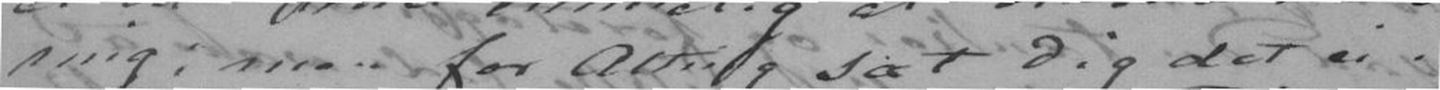mig, men for Alting sæt Dig det ei i
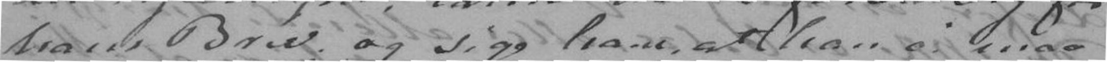hans Brev, og sige ham at han ei maa
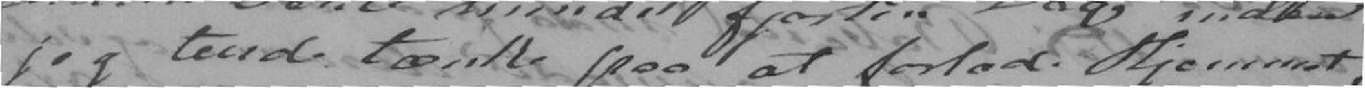jeg turde tænke paa at forlade Hjemmet,
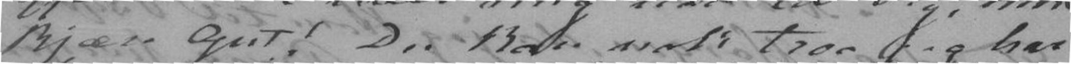kjære Gut! Du kan nok troe jeg har
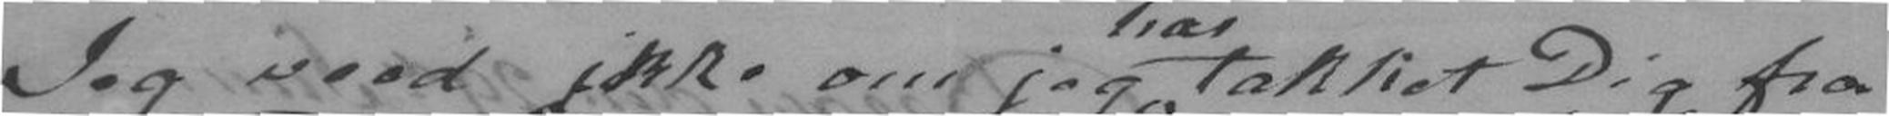Jeg veed ikke om jeg takket Dig fra
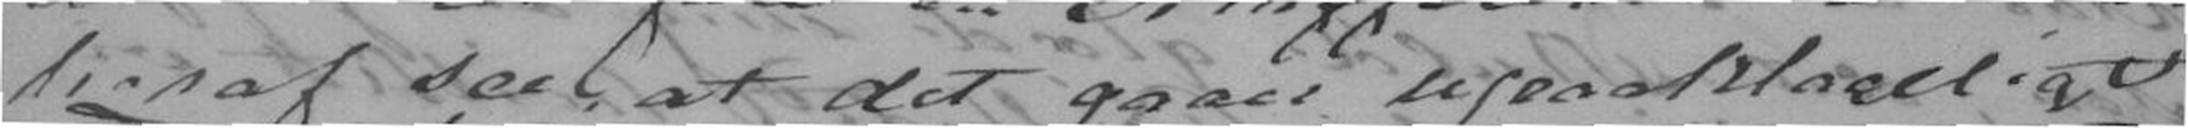heraf see, at det gaaer upaaklgeligt
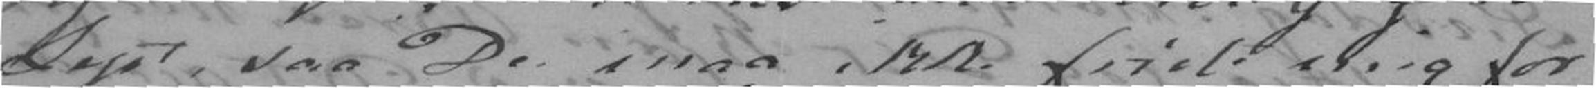Lyst, saa Du maa ikke friste mig for
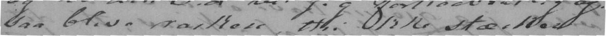saa blive raskere, thi ikke stærkere
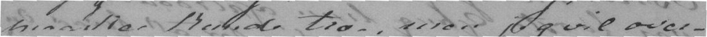maaskee kunde troe, men jeg vil over-
